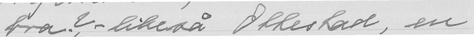bra? - likeså Ottestad, en
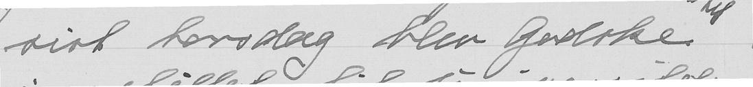sist torsdag blev Godske
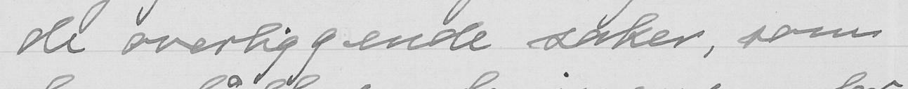de overliggende saker, som
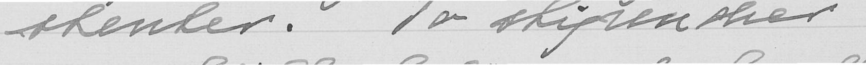stenter. To stipendier
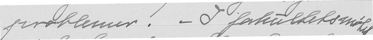problemer. - I fakultetsmøtet
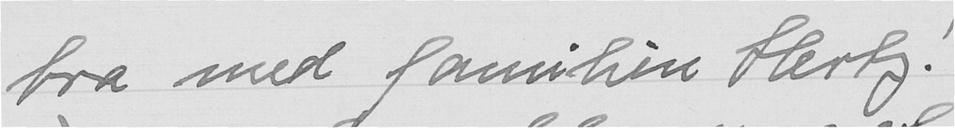bra med familien Hertz!
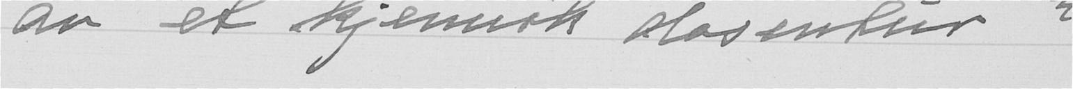av et kjemisk dosentur
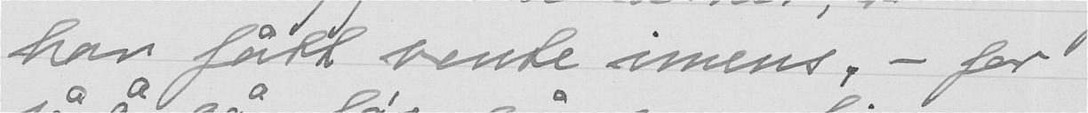har fått vente imens, - for
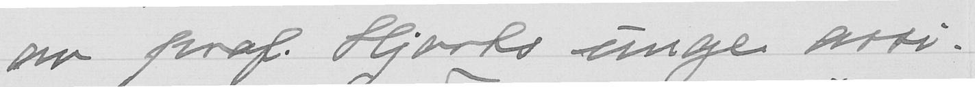av prof. Hjorts unge assi-
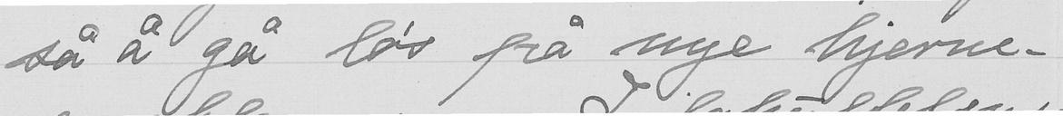så å gå løs på nye hjerne-
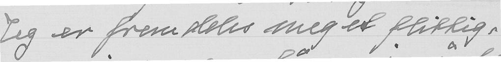Jeg er fremdeles meget flittig,
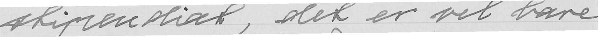stipendiat, det er vel bare
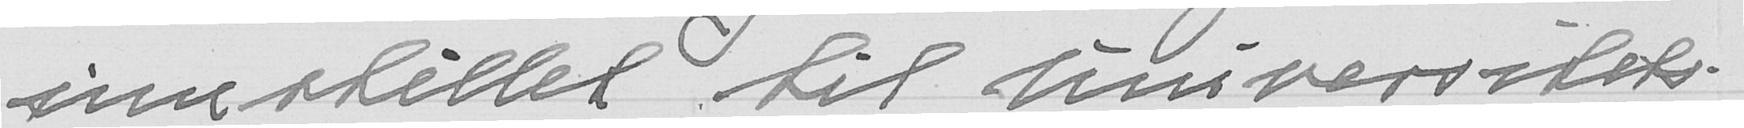innstillet til universitets-
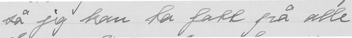så jeg kan ta fatt på alle
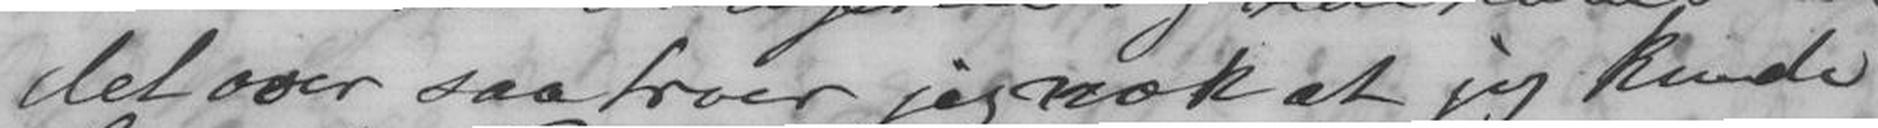det over saa troer jeg nok at jeg kunde
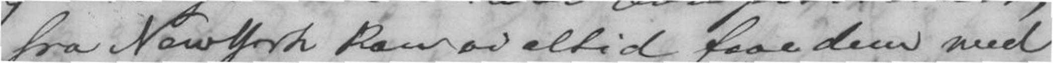fra New York kan vi altid faae dem med
